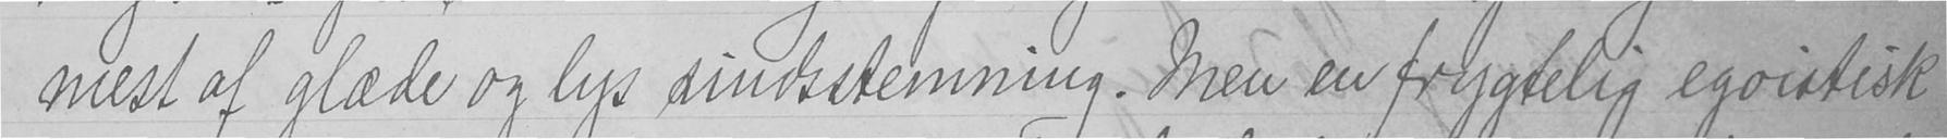mest af glæde og lys sindsstemning. Men en frygtelig egoistisk
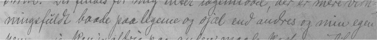ningsfuldt baade paa legeme og sjæl end andres og min egen
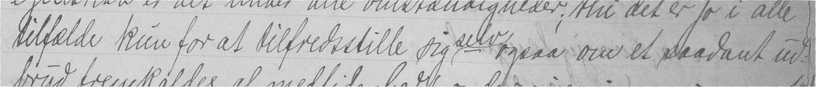tilfælde kun for at tilfredsstille sig selv ogsaa om et saadant ud-
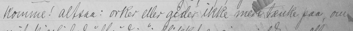komme! altsaa: orker eller gider ikke mere tænke paa, om
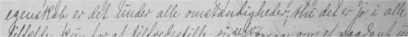egenskab er det under alle omstendigheder; Thi det er jo i alle
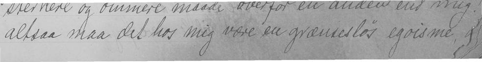altsaa maa det hos mig være en grænsesløs egoisme,
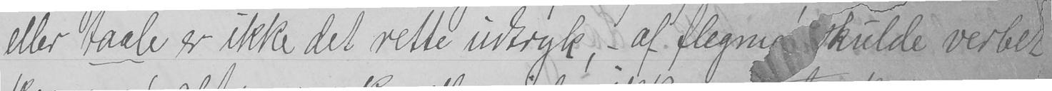eller taale er ikke det rette udtryk, - af flegme skulde verbet
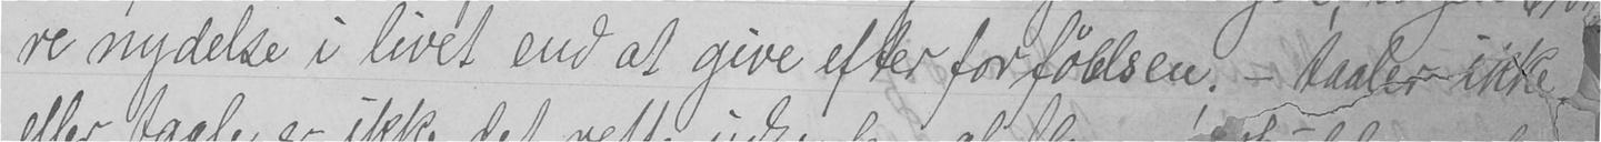re nydelse i livet end at give efter for følelsen; - Taaler ikke
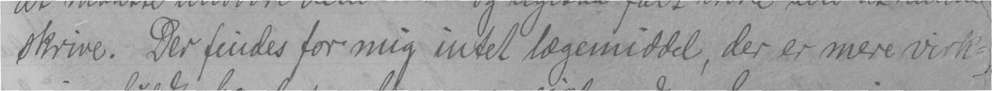skrive. Der findes for mig intet lægemiddel, der er mere virk-
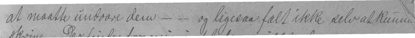at maatte undvære dem - - og ligesaa fælt ikke selv at kunne
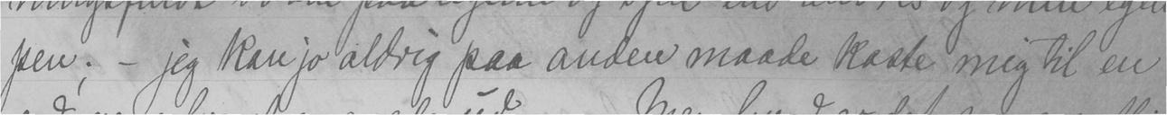pen; - jeg kan jo aldrig paa anden maade kaste mig til en
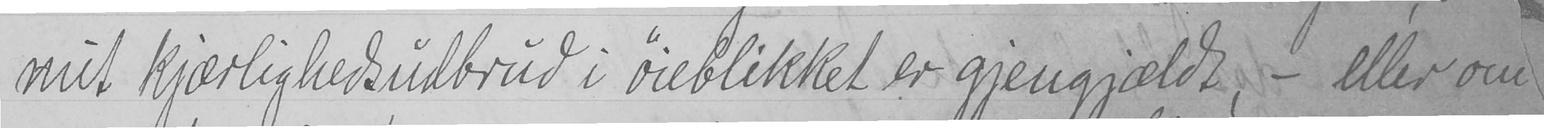mit kjærlighedsudbrud i øieblikket er gjengjældt, -  eller om
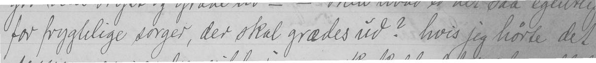for frygtelige sorger, der skal grædes ud? hvis jeg hørte det
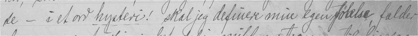se - i et ord hysteri! skal jeg definere min egen følelse, falder
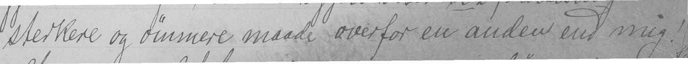sterkere og ømmere maade overfor en anden end mig!
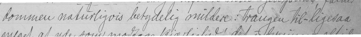dommen naturligvis betydelig mildere: Trangen til ligesaa
